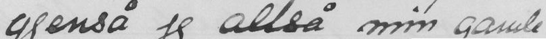gjenså jeg altså min gamle
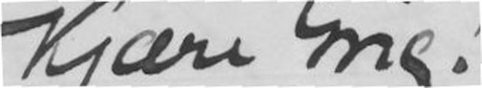Kjære Grieg!
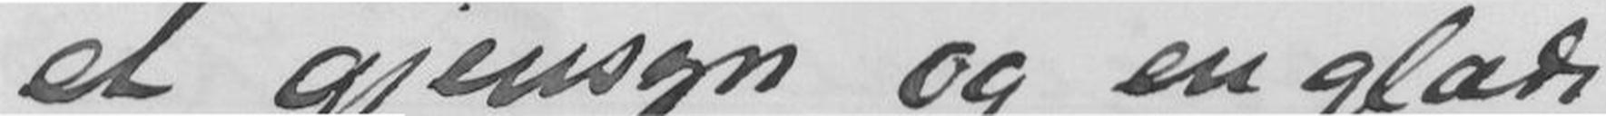et gjensyn og en glæde
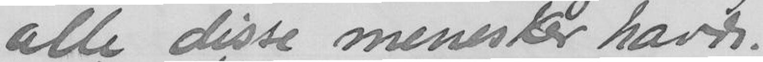alle disse mennesker havde.
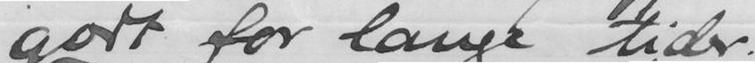godt for lange tider.
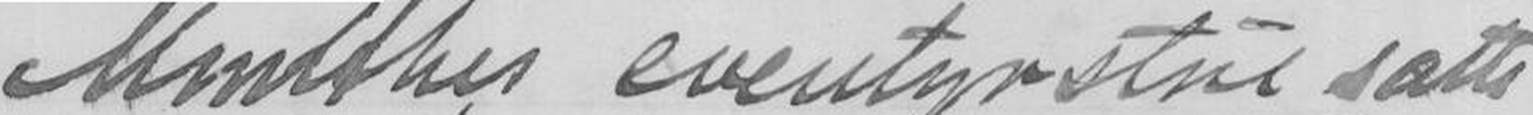Munthes eventyrstue satte
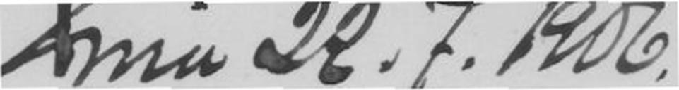Xnia 22.7.1906.
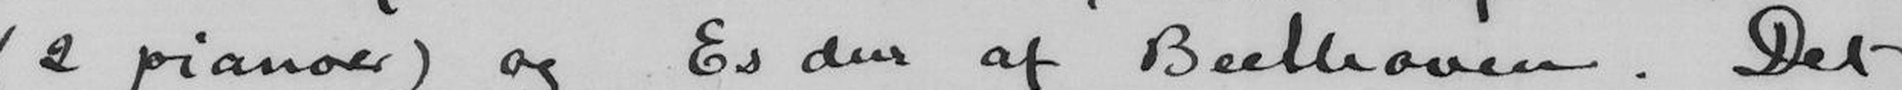(2 pianoer) og Es dur af Beethoven. Det
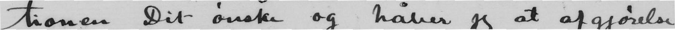tionen Dit ønske og håber jeg at afgjørelse
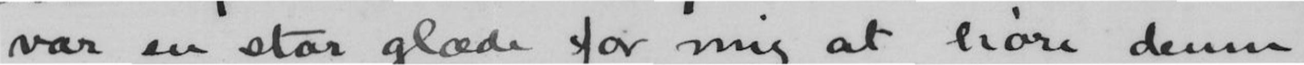var en stor glæde for mig at høre denne
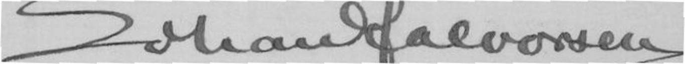Johan Halvorsen
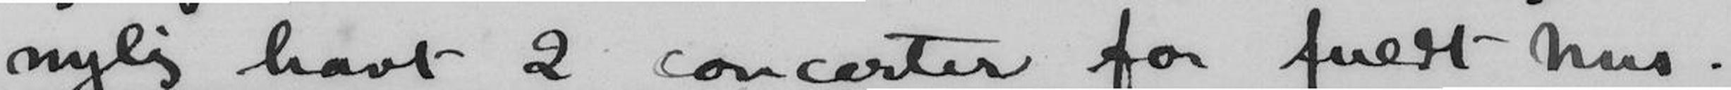nylig havt 2 concerter for fuldt hus.
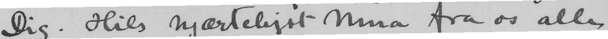Dig. Hils hjærteligst Nina fra os alle.
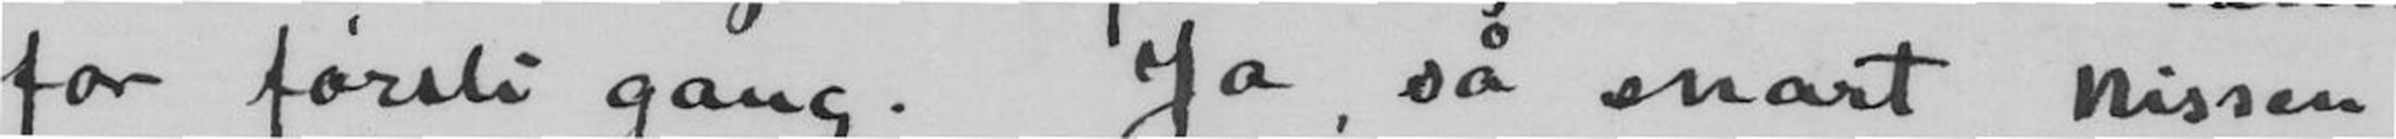for første gang. Ja, så snart Nissen
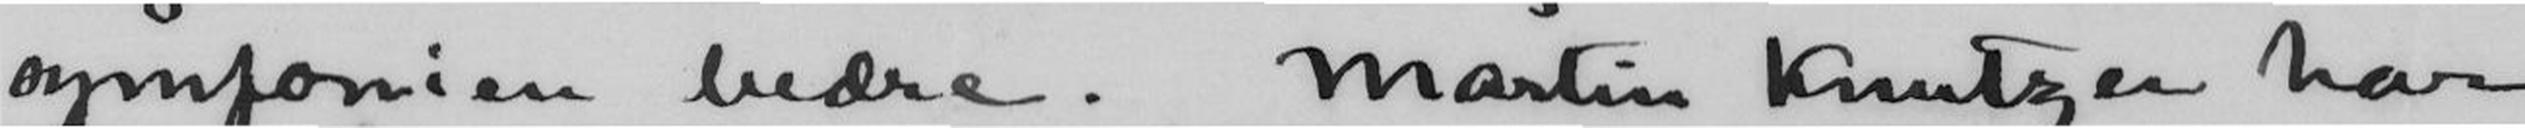symfonien bedre. Martin Knutzen har
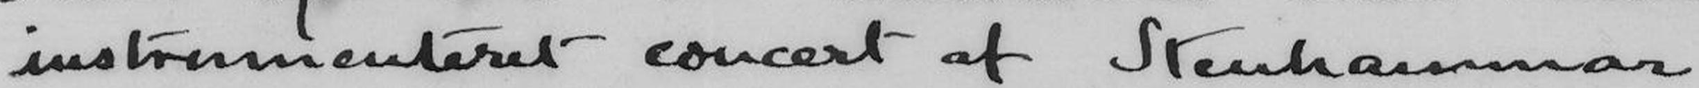instrumenteret concert af Stenhammar,
